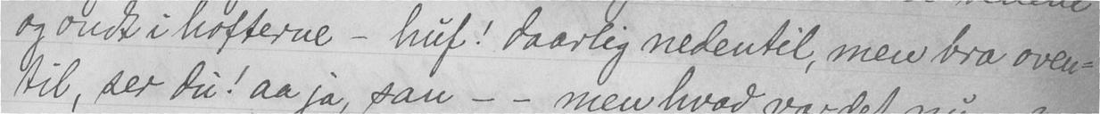og ondt i hofterne - huf! daarlig nedentil, men bra oven-
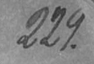229.
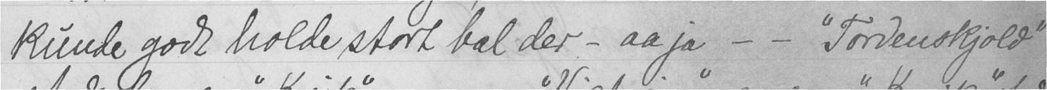kunde godt holde stort bal der - aa ja - - "Tordenskjold"
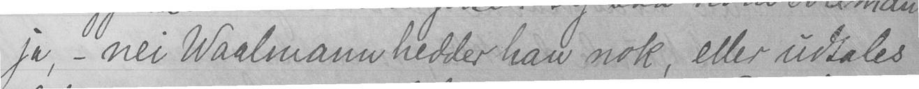Ja, - nei Waalmann hedder han nok, eller udtales
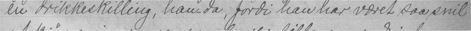en drikkeskilling, han da, fordi han har været saa snil
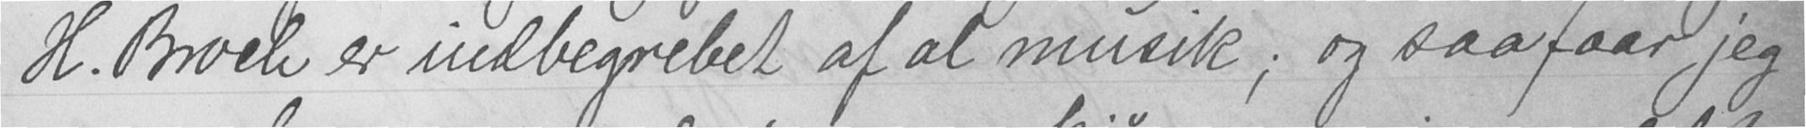H. Broch er indbegrebet af al musik; og saa faar jeg
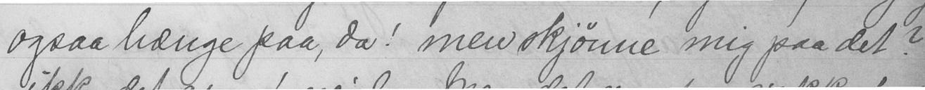ogsaa hænge paa, da! men skjønne mig paa det?
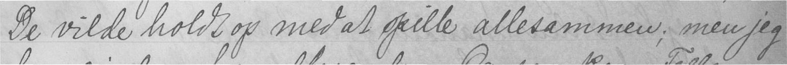De vilde holdt op med at spille allesammen; men jeg
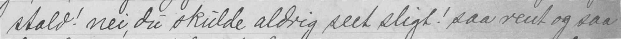stald! nei, du skulde aldrig seet sligt! saa rent og saa
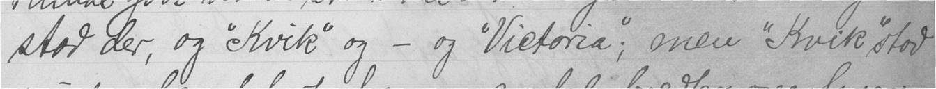stod der, og "Kvik" og - og "Victoria"; men "Kvik" stod
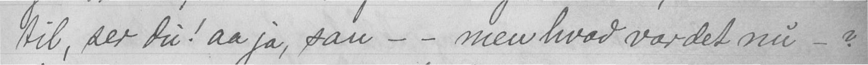til, ser du! aa ja, san - - men hvad var det nu - ?
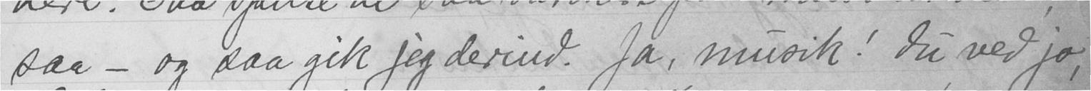saa - og saa gik jeg derind. Ja, musik! du ved jo,
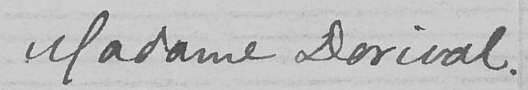Madame Dorival.
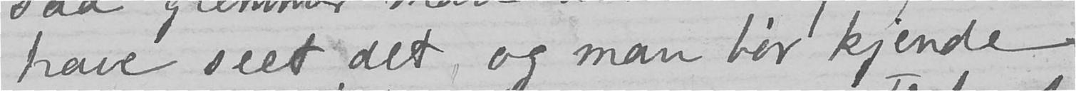have seet det, og man bør kjende
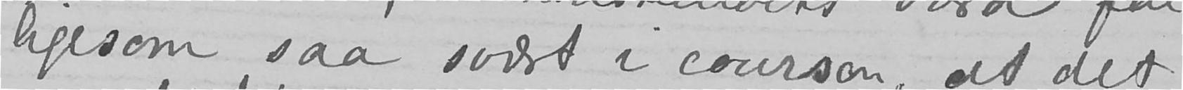ligesom saa svært i coursen, at det
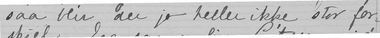saa blir der jo heller ikke stor for-
